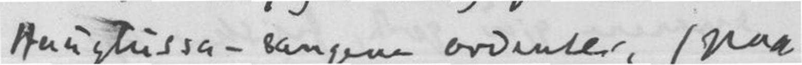Haugtussa-sangene ordentlig, (paa
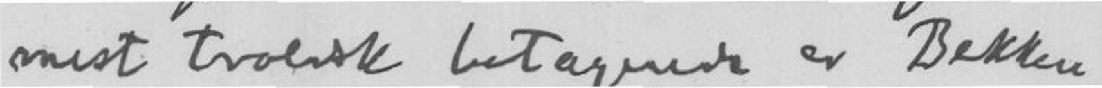mest trolsk betagende er Bekken
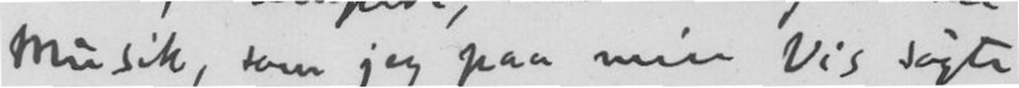Musik, som jeg paa min Vis søgte
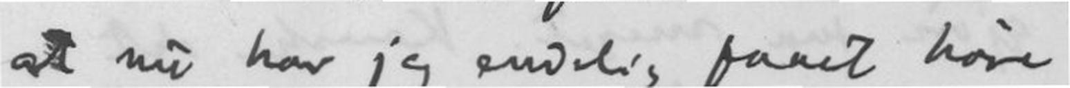at nu har jeg endelig, faaet høre
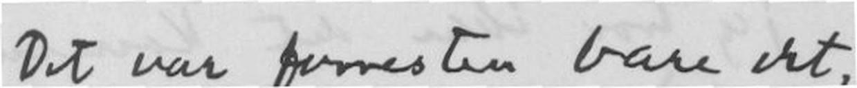Det var forresten bare det,
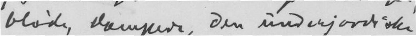bløde, Dæmpede, den underjordiske
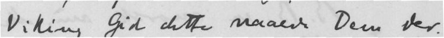Viking. Gid dette naaede Dem der.
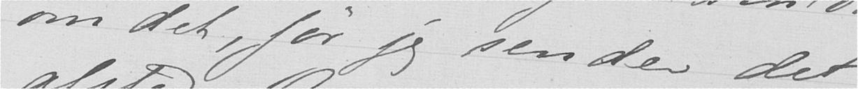om det, før jeg sender det
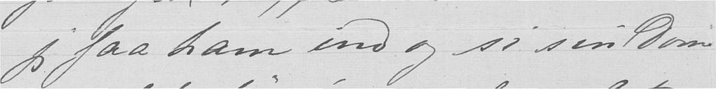jeg faa ham ind og si sin Dom
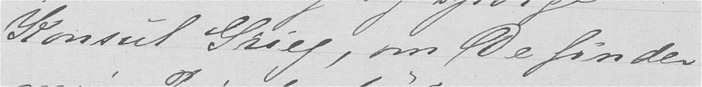Konsul Grieg, om De finder
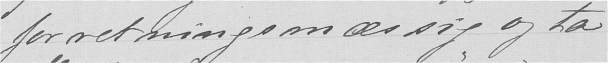forretningsmæssig og ta
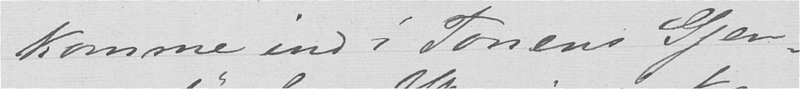komme ind i Tonens Gjen-
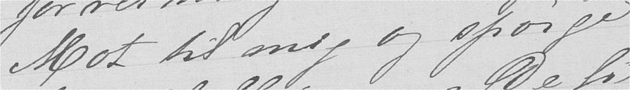Mot til mig og spørge
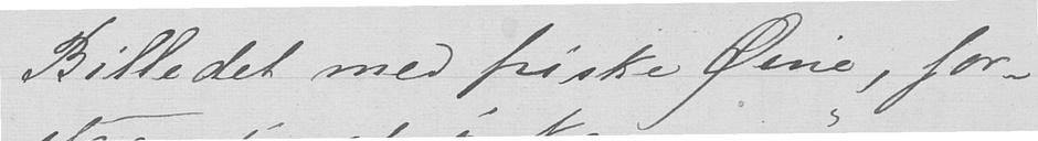Billedet med friske Øine, for-
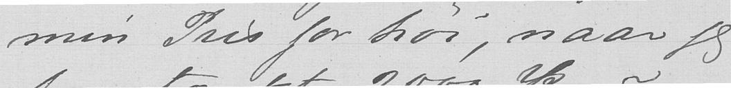min Pris for høi, naar jeg
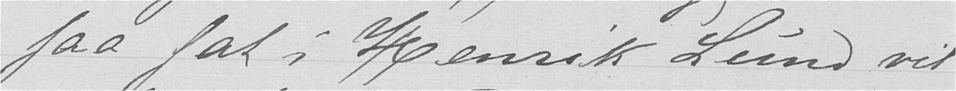faa fat i Henrik Lund vil
